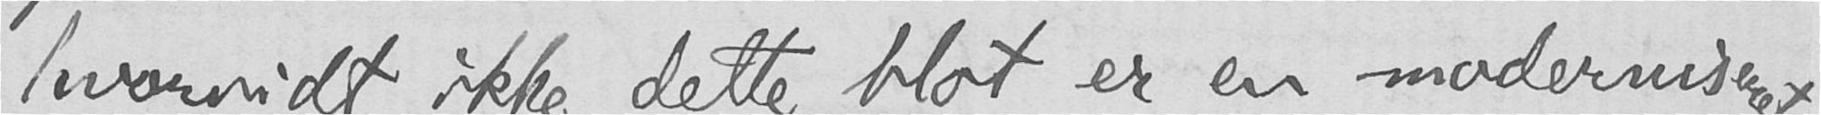hvorvidt ikke dette blot er en moderniseret
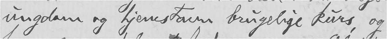ungdom og hjemstavn brugelige kurs, og
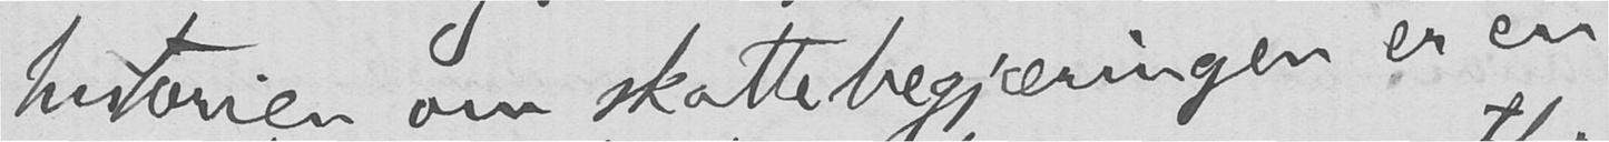historien om skattebegjæringen er en
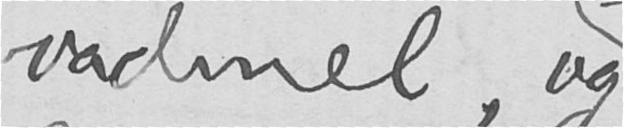vadmel, og
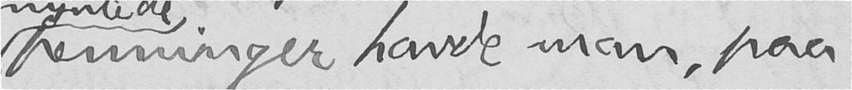penninger havde man, paa
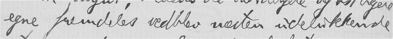egne fremdeles vedblev næsten udelukkende
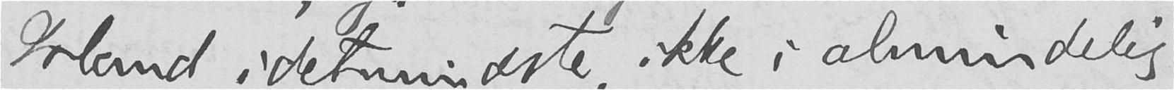Island idetmindste, ikke i almindelig
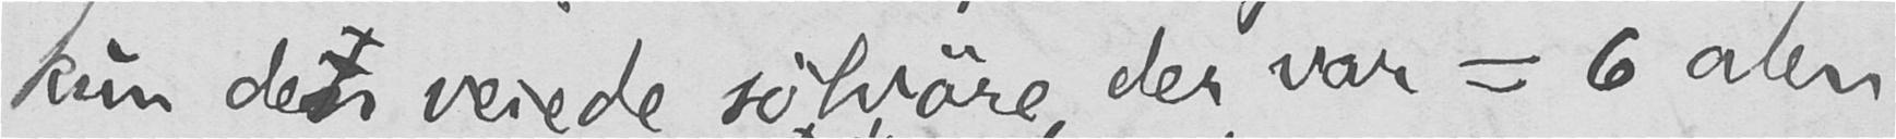kun det veiede sølvøre, der var = 6 alen
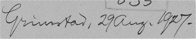Grimstad, 29Aug. 1927.
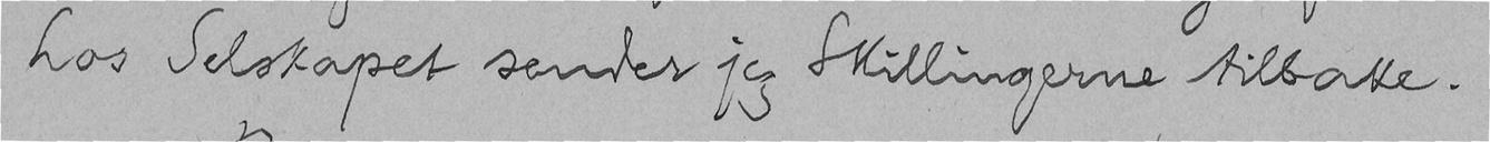hos Selskapet sender jeg Skillingerne tilbake.
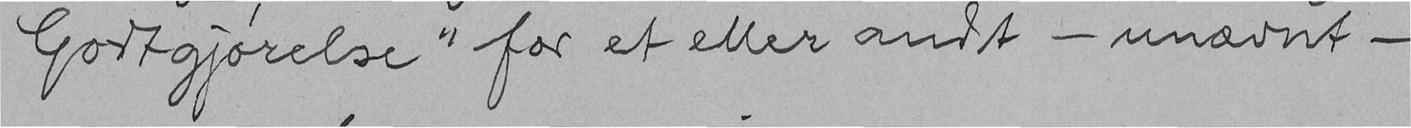Godtgjørelse" for et eller andet - unævnt -
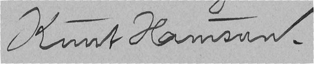Knut Hamsun.
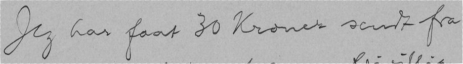Jeg har faat 30 Kroner sendt fra
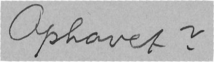Ophavet?
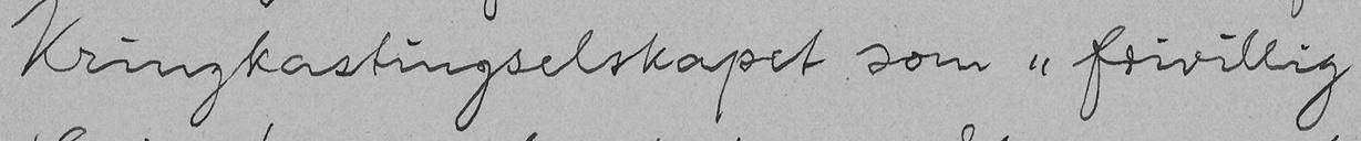Kringkastingselskapet som "frivillig
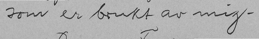som er brukt av mig.
